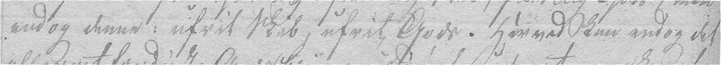endog denne: ufrit Skib, ufrit Gods. Herved kan endog det
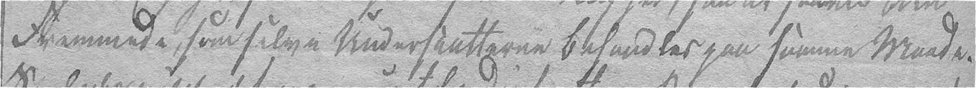Fremmede som selve Undersaatterne behandles paa samme Maade.
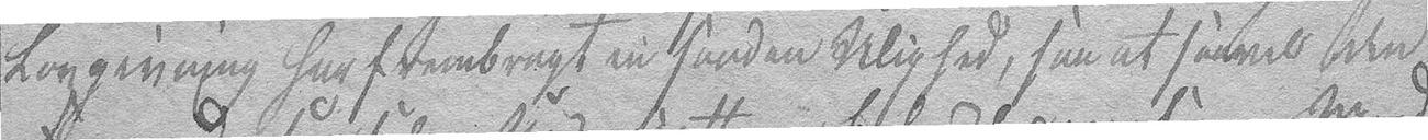Lovgivning har frembragt en saadan Ulighed, saa at saavel den
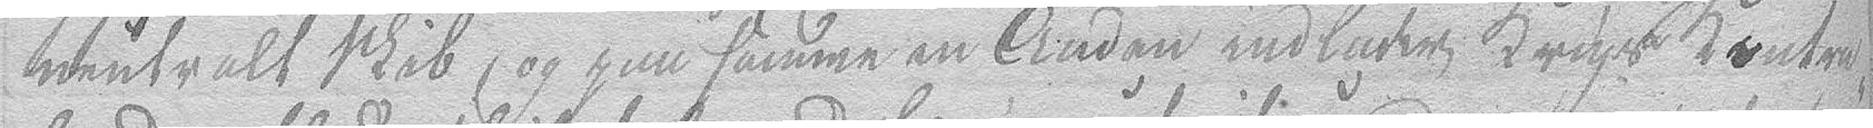neutralt Skib, og paa samme en Anden indlader Krigs Kontra-
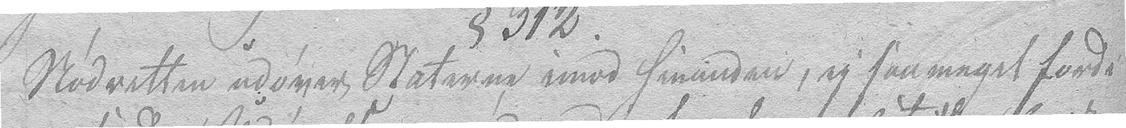Nødretten udøver Staterne imod hinanden, ey saa meget fordi
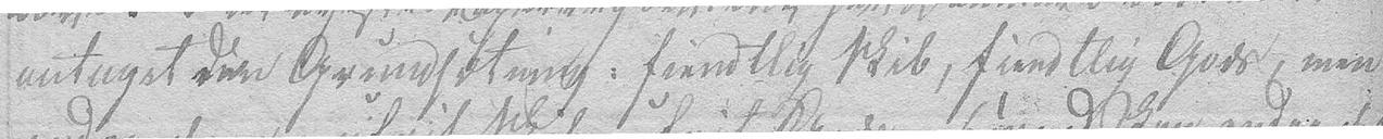antaget den Grundsætning: fiendtlig Skib, fiendtlig Gods, men
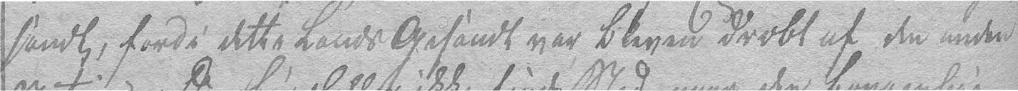sandt, fordi dette Lands Gesandt var bleven dræbt af den anden
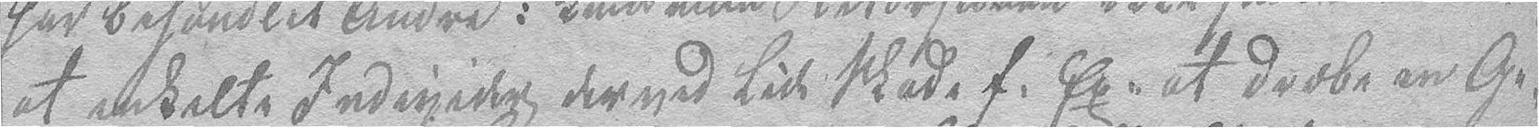at enkelte Individer derved lide Skade f: Ex: at dræbe en Ge-
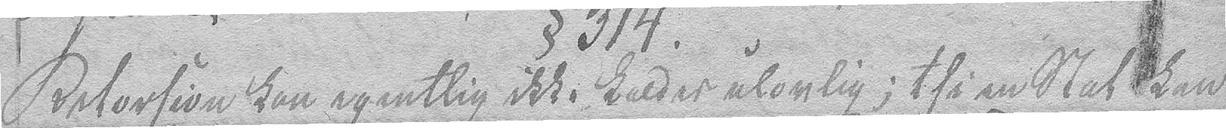Retorsion kan egentlig ikke kaldes ulovlig; thi en Stat kan
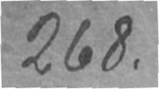268.
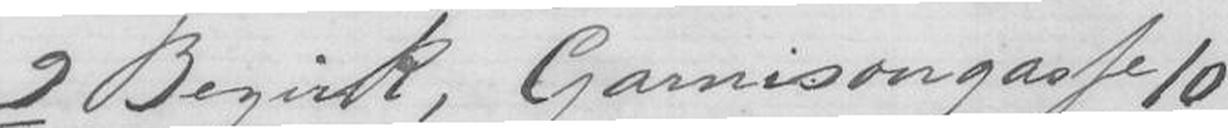I Begirk, Garnisongasse 10
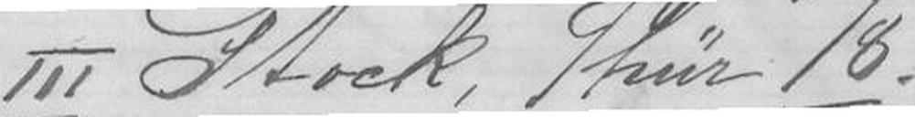III Stock, Fhür 18.
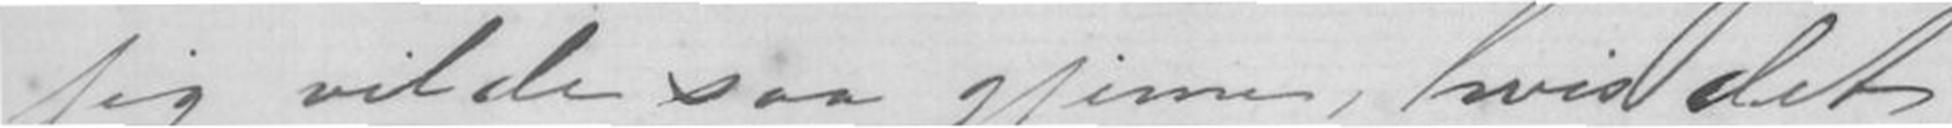jeg vilde saa gjerne, hvis det
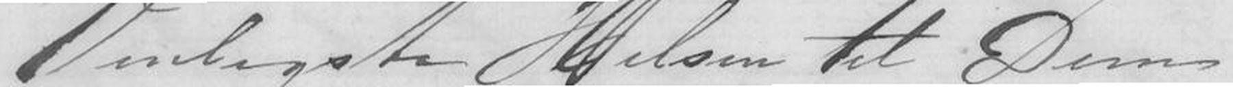Venligste Hilsen til Dem
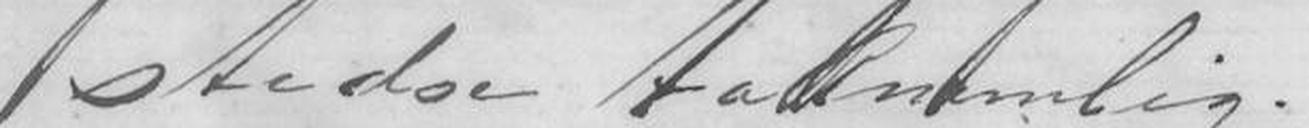stedse taknemlig.
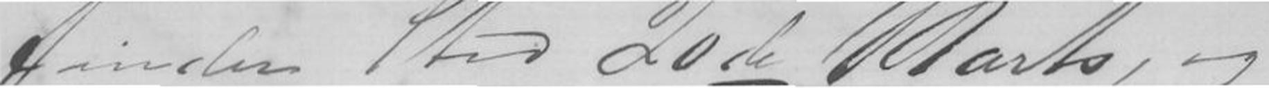finder Sted 20de Marts, og
